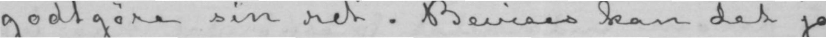godtgøre sin ret. Bevises kan det jo
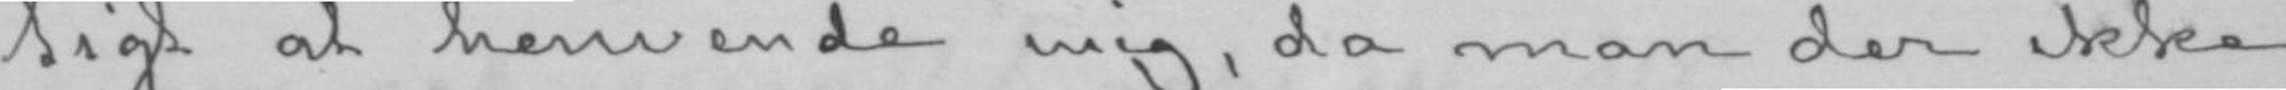tigt at henvende mig, da man der ikke
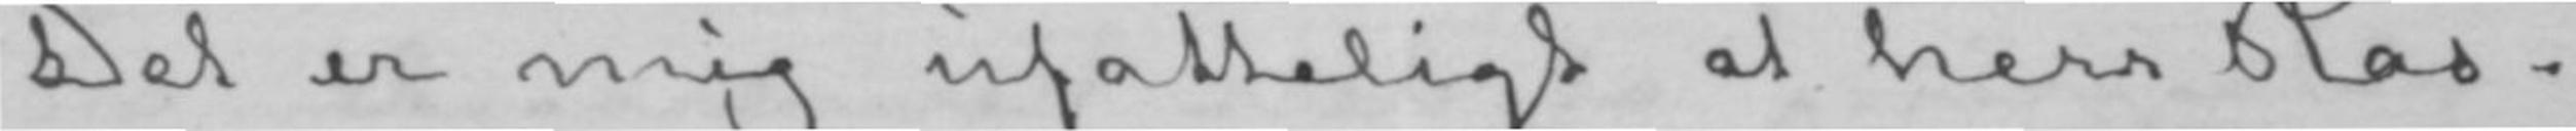Det er mig ufatteligt at herr Ras-
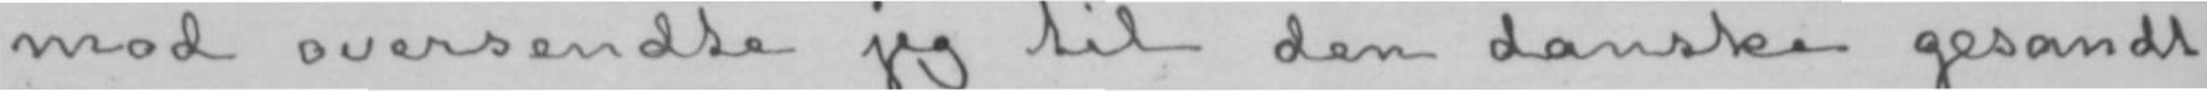mod oversendte jeg til den danske gesandt
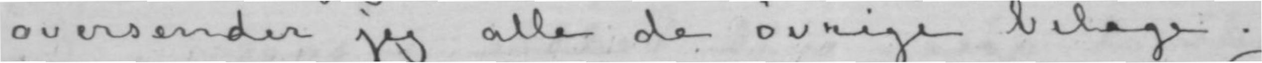oversender jeg alle de øvrige bilage.
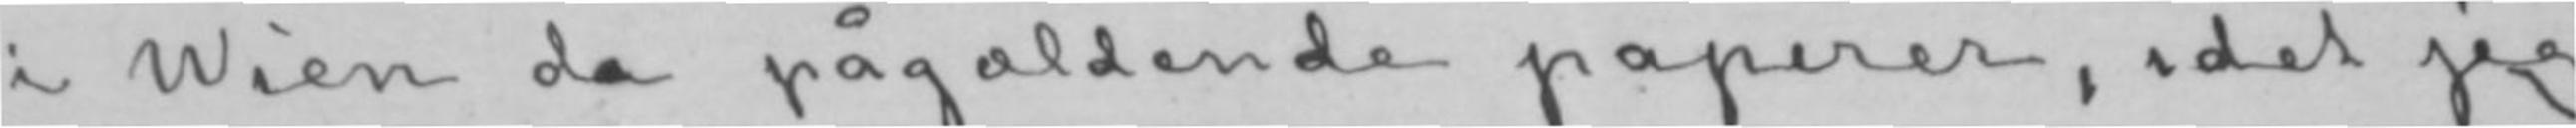i Wien de pågældende papirer, idet jeg
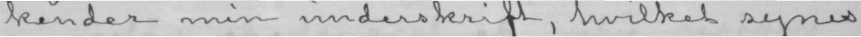kender min underskrift, hvilket synes
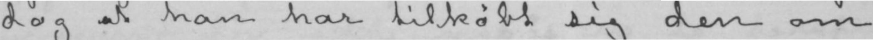dog at han har tilkøbt sig den om-
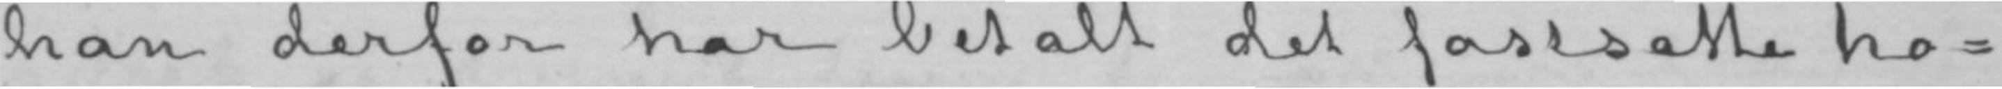han derfor har betalt det fastsatte ho-
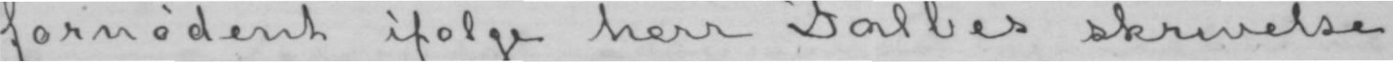fornødent ifølge herr Falbes skrivelse,
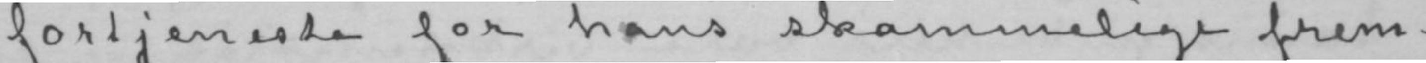fortjeneste for hans skammelige frem-
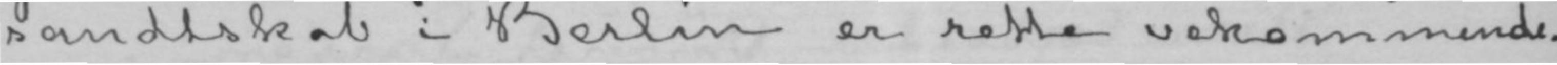sandtskap i Berlin er rette vedkommende.
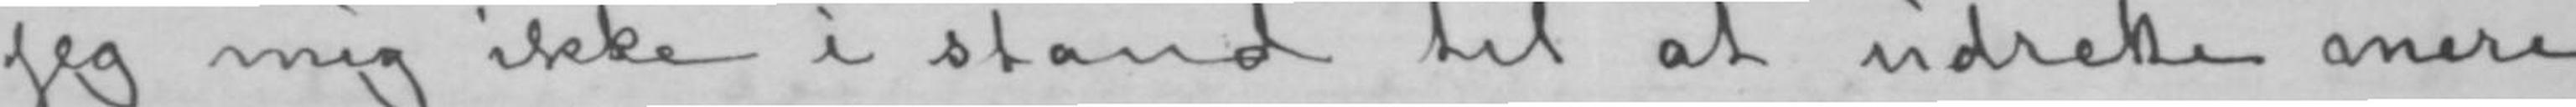jeg mig ikke i stand til at udrette mere
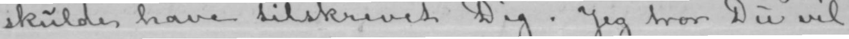skulde have tilskrevet Dig. Jeg tror Du vil
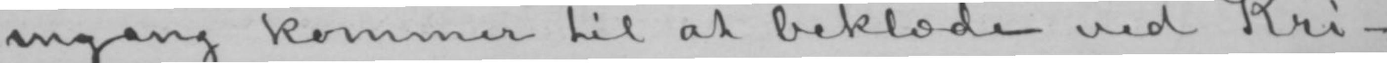engang kommer til at beklæde ved Kri-
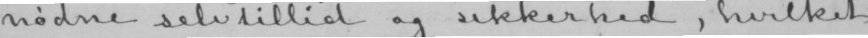nødne selvtillid og sikkerhed, hvilket
